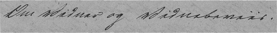Om Vidner og Vidnebeviis.
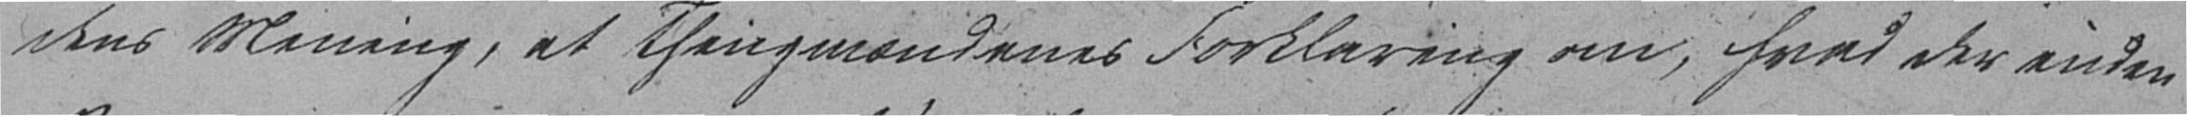dens Mening, at Thingmændenes Forklaring om, hvad der inden
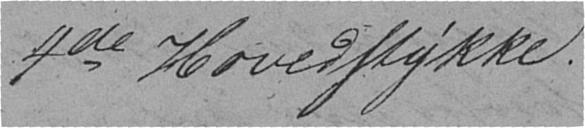4de Hovedstykke.
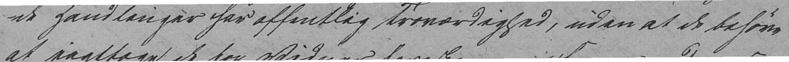de Handlinger har offentlig Troværdighed, uden at de behøve
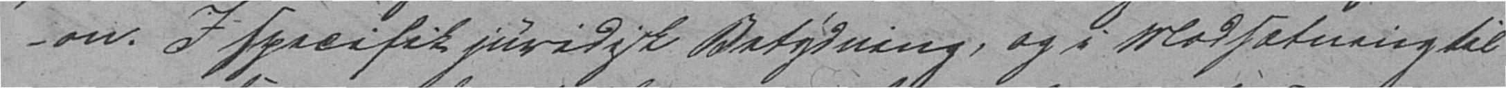on. I specifik juridisk Betydning, og i modsætning til
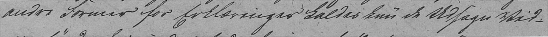andre Former for Erklæringer kaldes kun de Udsagn Vid-
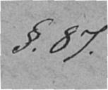§. 87.
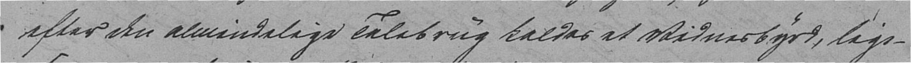efter den almindelige Talebrug kaldes et Vidnesbyrd, lige-
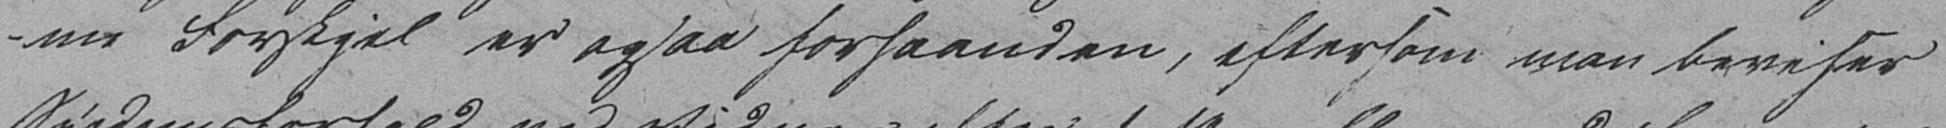me Forskjel er ogsaa forhaanden, eftersom man beviser
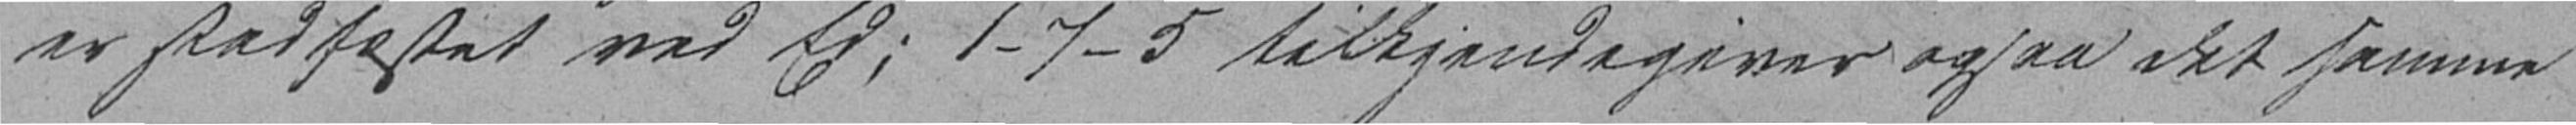er stadfæstet ved Ed; 1-7-5 tilkjendegiver ogsaa det samme
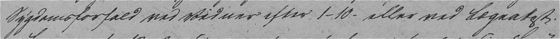Sygdomsforfald ved Vidner efter 1–10 - eller ved Lægeattest.
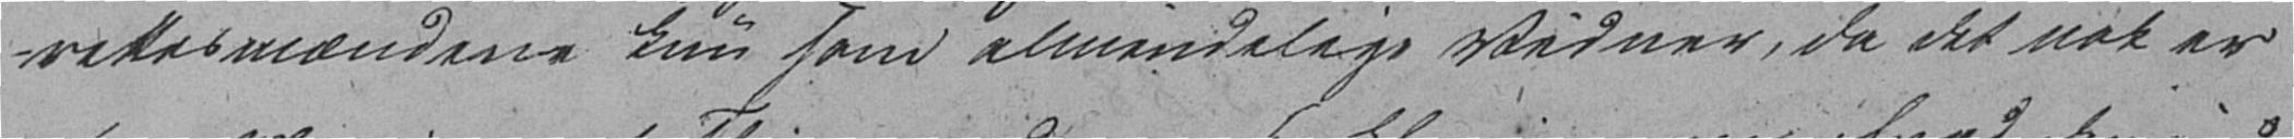rettesmændene kun som almindelige Vidner, da det nok er
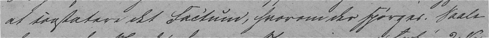at constatere det Factum, hvorom der spørges. Saale-
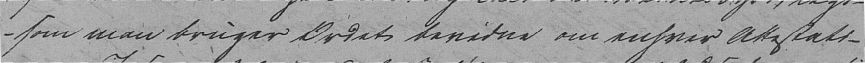som man bruger Ordet bevidne om enhver Attestati-
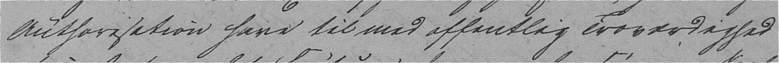Authorisation have til med offentlig Troverdighed
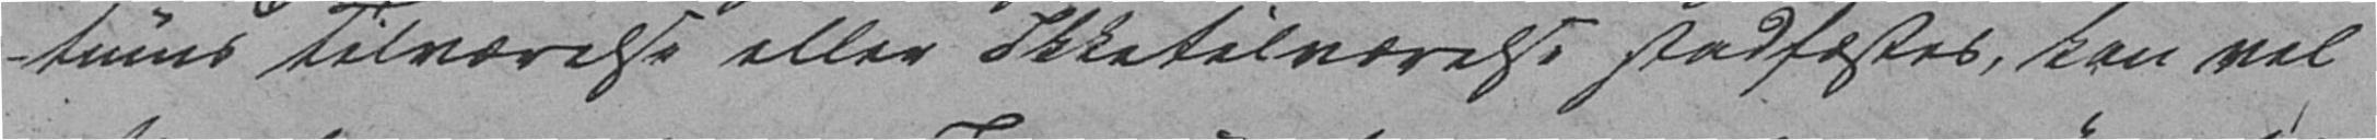tums Tilværelse eller Ikketilværelse stadfæstes, kan vel
